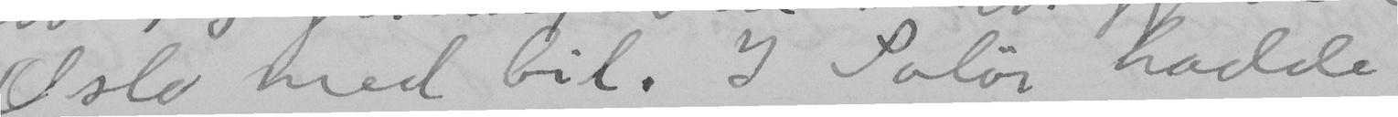Oslo med bil. I Solör hadde
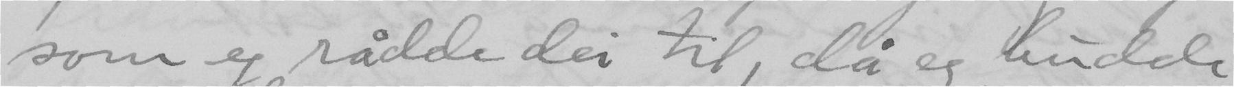som eg rådde dei til, då eg budde
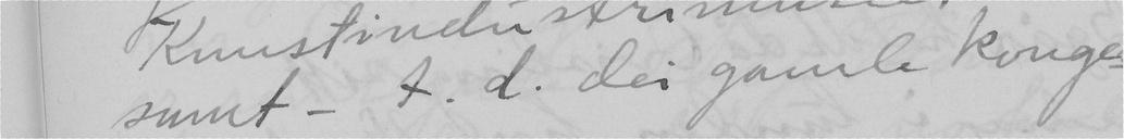sumt – t.d. dei gamle Konge-
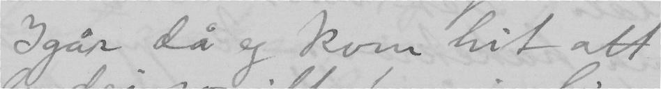Igår då eg kom hit att
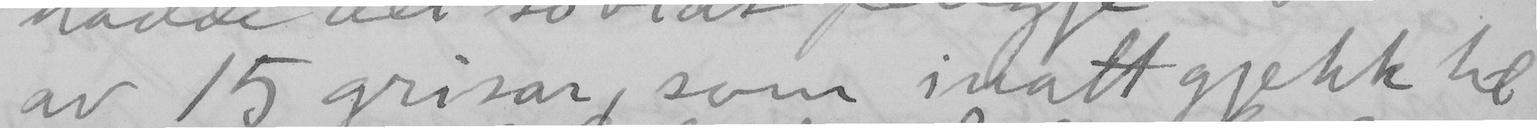av 15 grisar, som inatt gjekk til
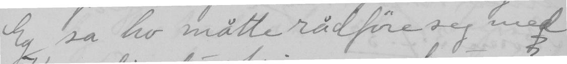Eg sa ho måtte rådföre seg med
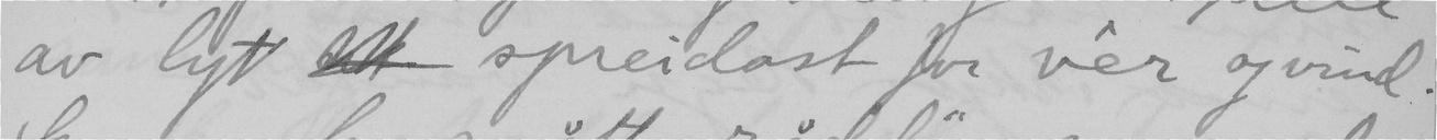av lyt  spreidast for vær og vind:
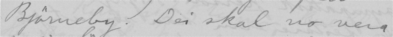Björneby. Dei skal no vera
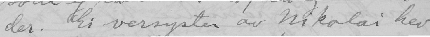der. Ei versyster av Nikolai hev
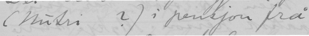(nutri?) i pensjon frå
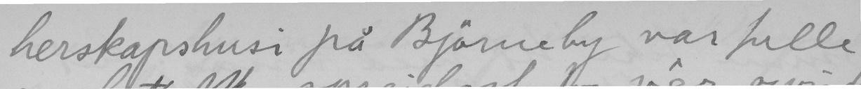herskapshusi på Björneby var fulle
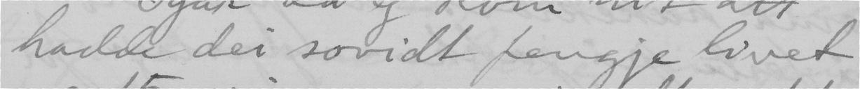hadde dei sovidt fengje livet
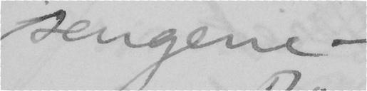sengene –
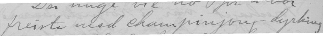freiste med champinjong-dyrking
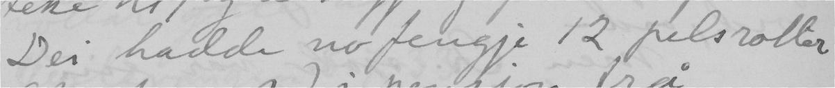Dei hadde no fengje 12 pelsroller
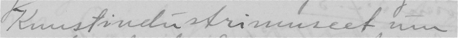Kunstindustrimuseet um
